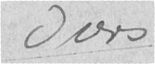Deres
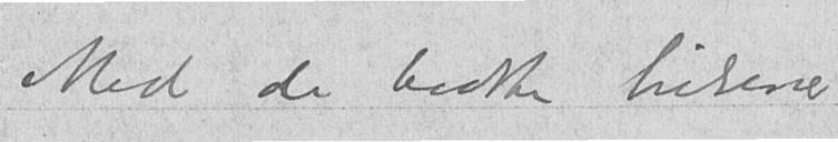Med de bedste hilsener
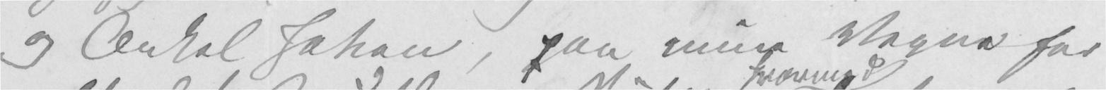og Onkel Johan, paa mine Vegne for
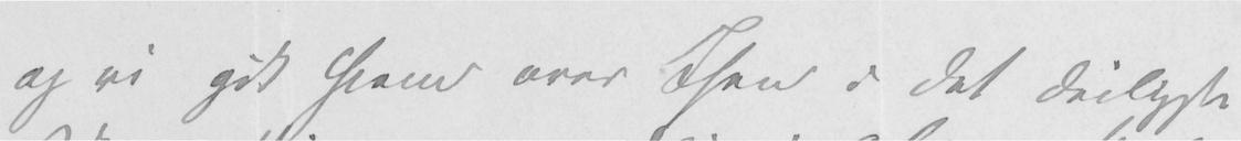og vi gik hjem over Isen i det deiligste
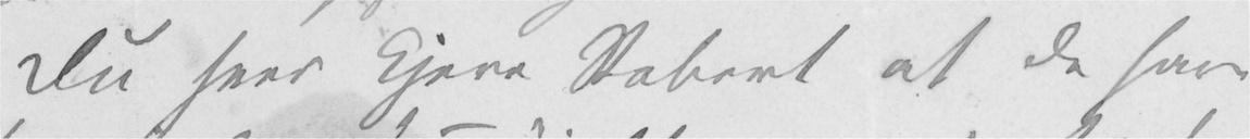Du seer kjere Robert at de have
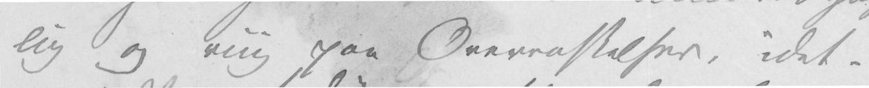lig og riig paa Overraskelser, idet-
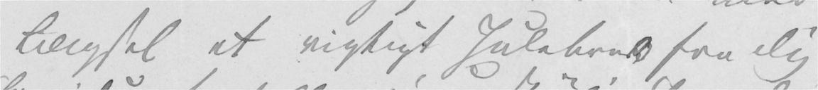Længsel et rigtigt Julebrev fra Dig
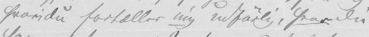hvori Du fortæller mig udførlig, hvor Du
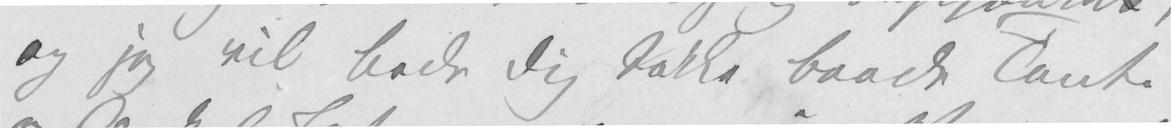og jeg vil bede Dig takke baade Tante
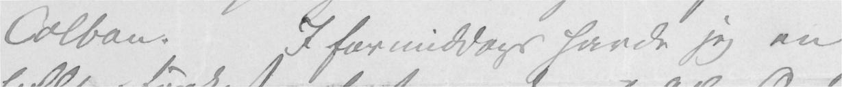Colban. Iformiddags havde jeg en
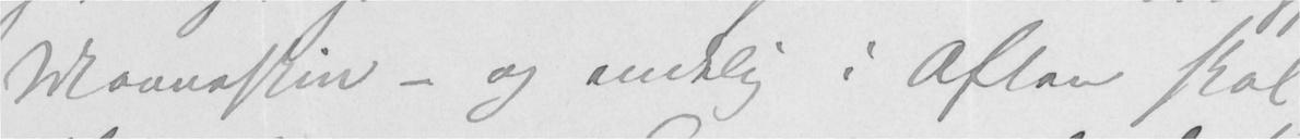Maaneskin – og endelig i Aften skal
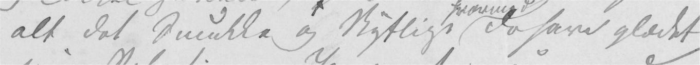alt det Smukke og Nyttige de have glædet
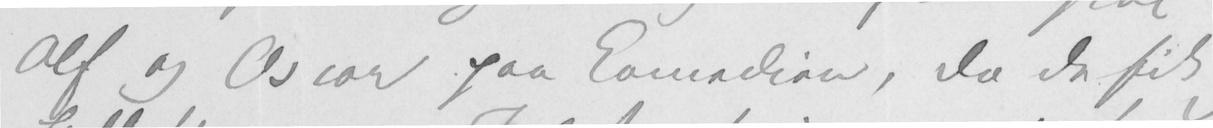Alf og Oscar paa Komedien, da de fik
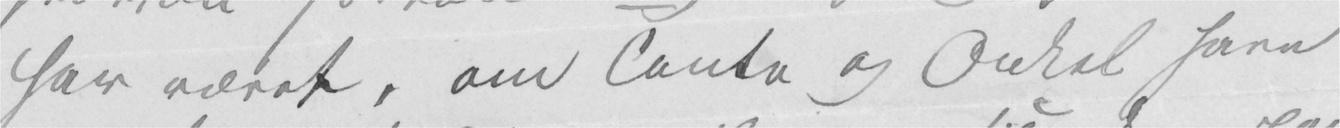har været, om Tante og Onkel have
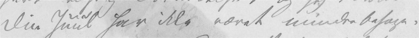Din Juul har ikke været mindre behage-
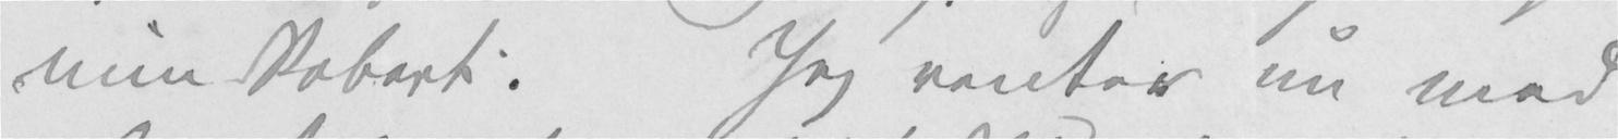min Robert. Jeg venter nu med
